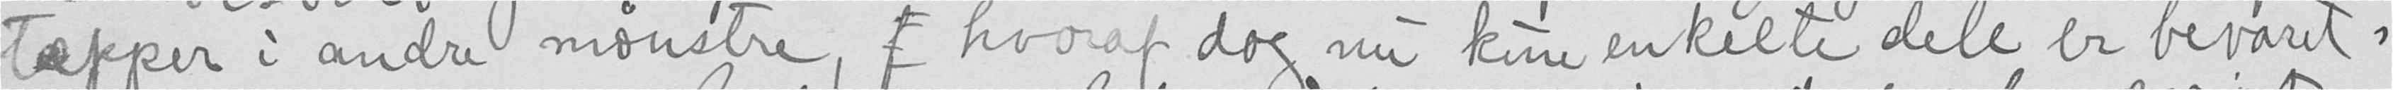tæpper i andre mønstre,  hvoaf dog nu kun enkelte dele er bevart.
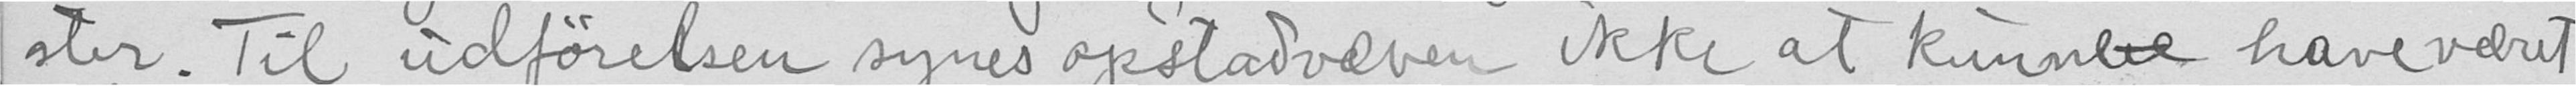ster. Til udførelsen synes opstadvæven ikke at kunne have været
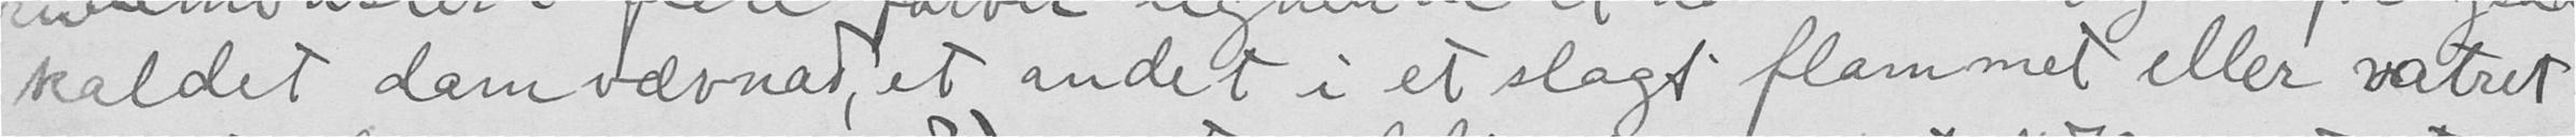kaldet dem vævnad, et andet i et slagt flammet eller vatret
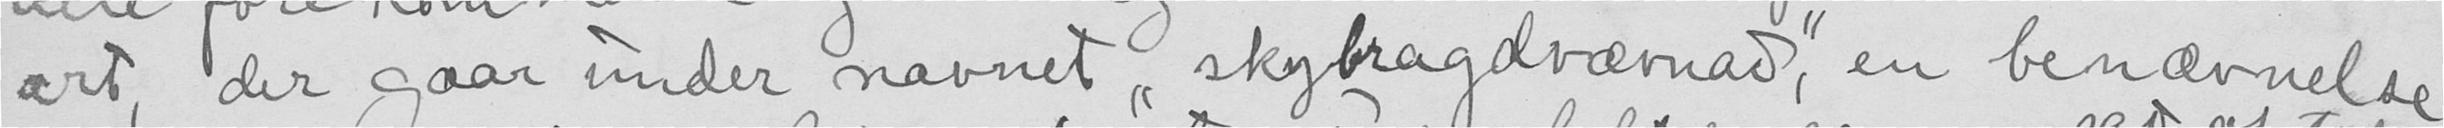art, der gaar under navnet "skybragdvævnad", en benævnelse
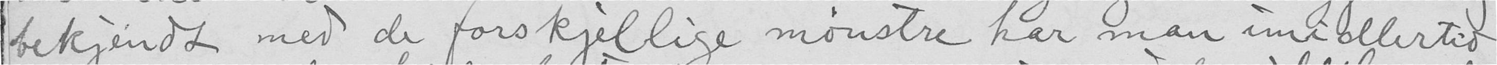bekjent med de forskjellige mønstre har man imidlertid
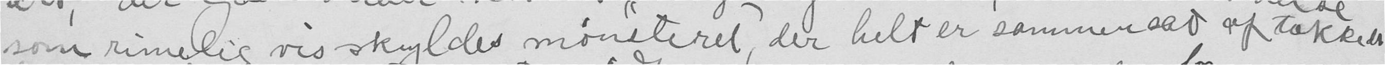som rimeligvis skyldes mønsteret der helt er sammensat af takkers
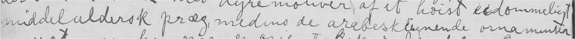middelaldersk præg medens de arabesklignende ornamenter
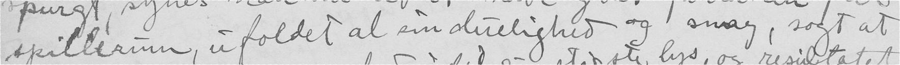spillerum, ufoldet al sin duelighed og smag, sagt at
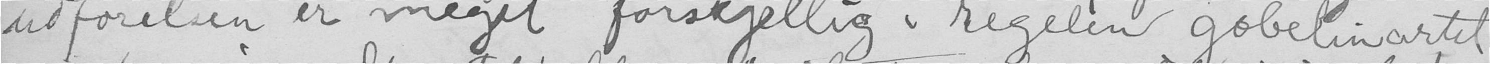udførelsen er meget forskjellig i regelen gobelin artet
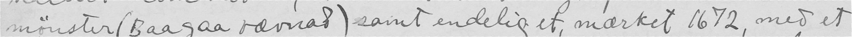mønster (Baagaa vævnad) samt endelig et, mærket 1672, med et
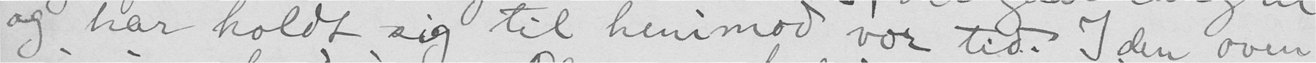og har holdt sig til henimod vor tid. Iden oven
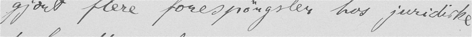gjort flere forespørgsler hos juridiske
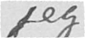jeg
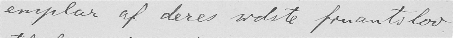emplar af deres sidste finantslov
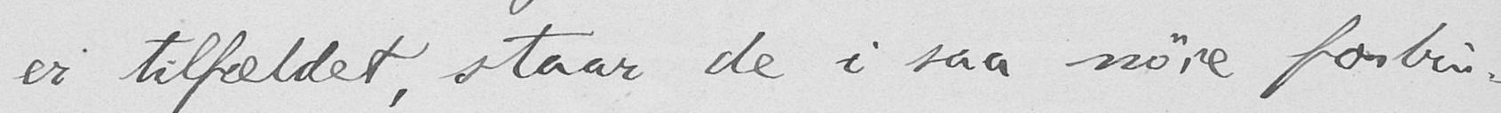er tilfældet, staar de i saa nøie forbin-
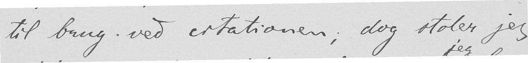til brug ved citationen; dog stoler jeg
